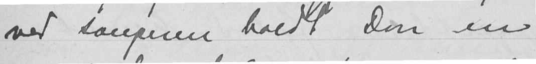ved souperen holdt Don en
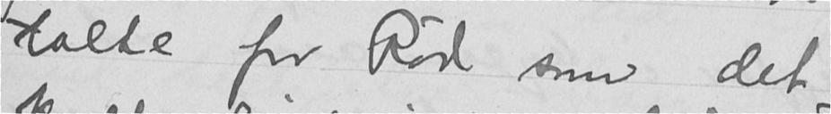talte for Rød som det
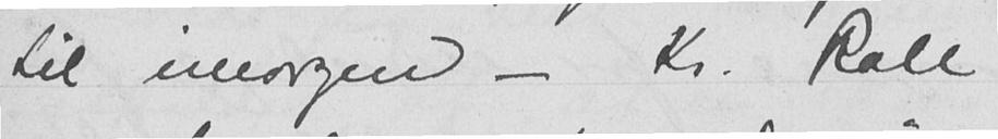til imorgen - Kr. Roll
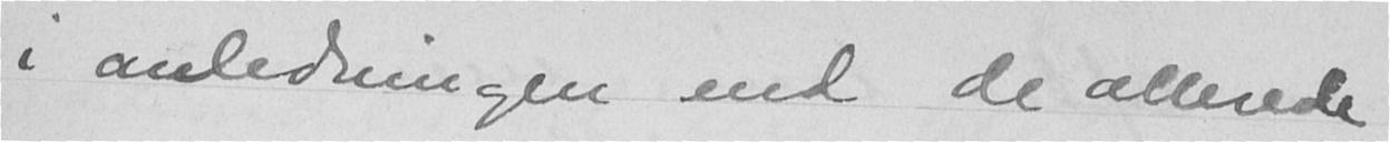i anledningen end de allerede
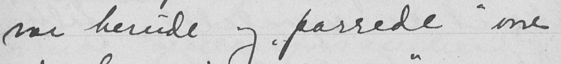var herude og passede vore
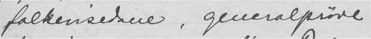folkevisedans, generalprøve
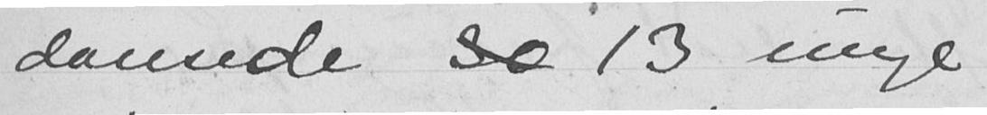dansede 30 13 unge
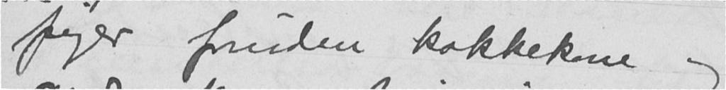piger foruden kokkekone og
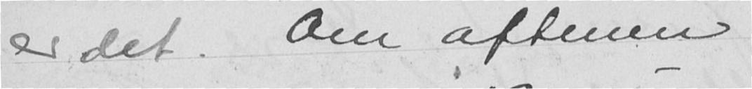er det. Om aftenen
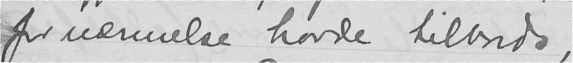fornærmelse havde tilbords,
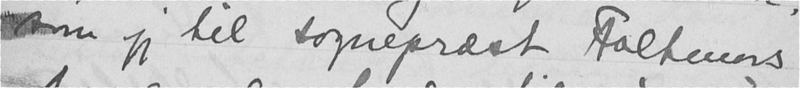som jeg til sognepræst Foltmars
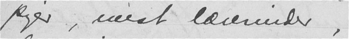piger, mest lærerinder,
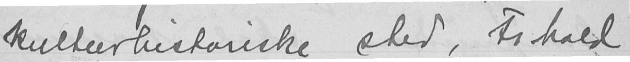kulturhistoriske sted, Fr hald
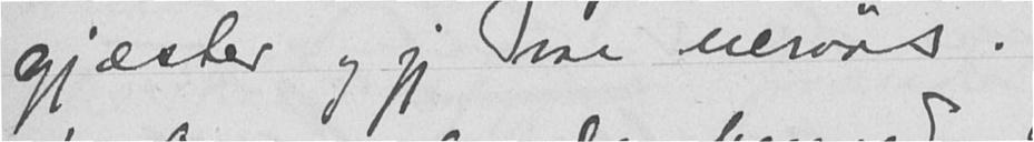gjæster og jeg var nervøs.
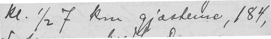Kl. ½ 7 kom gjæsterne, 184,
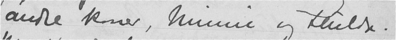andre koner, Minni og Hulda.
